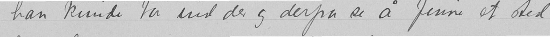han kunde ta ind der og derfra se å finne et sted
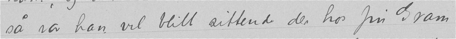så var han vel blitt sittende der hos fru Gram
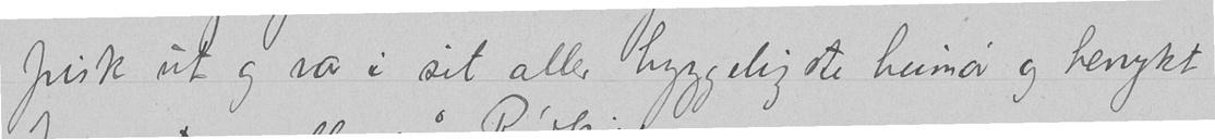frisk ut og var i sit aller hyggeligste humør og henrykt
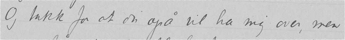Og takk for at du også vil ha mig over, men
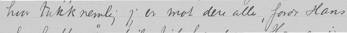hvor takknemlig jeg er mot dere alle, fordi Hans
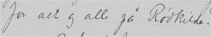for alt og alle på Rødkilde.
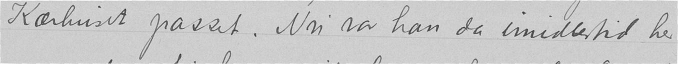Kærhuset passet. Nu var han da imidlertid her
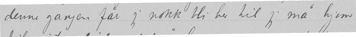denne gangen får jeg nokk bli her til jeg må hjem
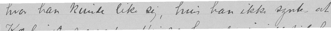hvor han kunde like sig, hvis han ikke syntes at
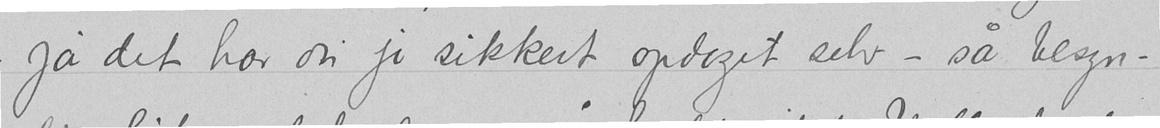– ja det har du jo sikkert opdaget selv – så besyn-
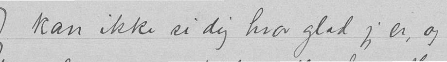Jeg kan ikke si dig hvor glad jeg er, og
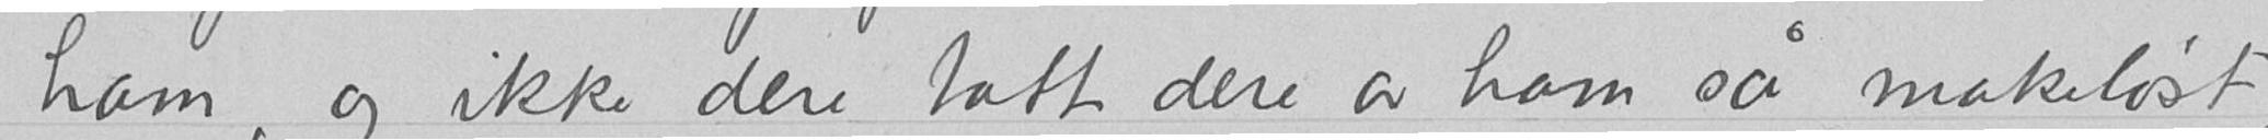ham, og ikke dere tatt dere av ham sa makeløst,
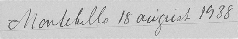Montebello 18 august 1938
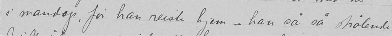i mandags, før han reiste hjem – han så så strålende
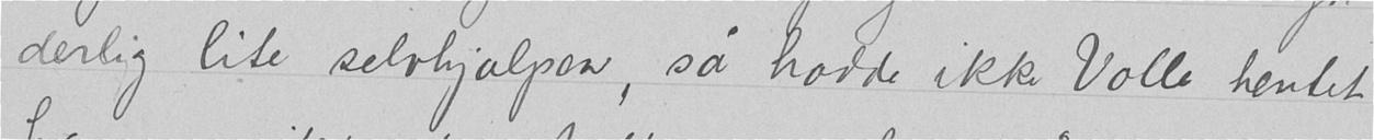derlig lite selvhjulpen, så hadde ikke Volle hentet
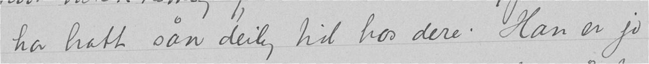har hatt sån deilig tid hos dere. Han er jo
